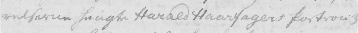relserne hengte Harald Haarfagers Portrait
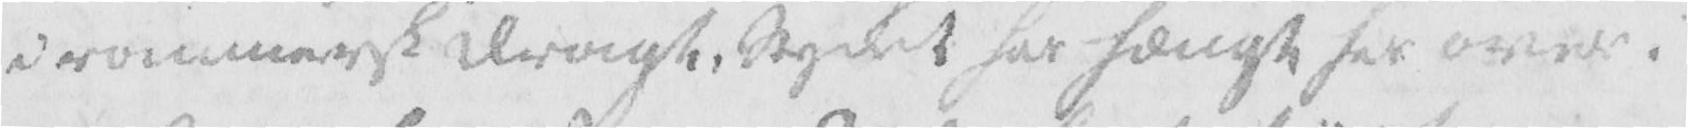i rommersk Dragt. Stycket har hængt her over i
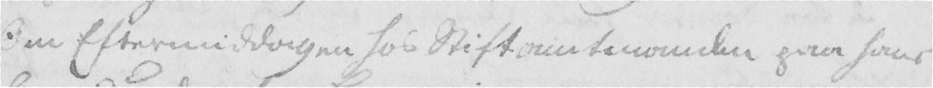Om Eftermiddagen hos Stiftamtmanden paa hans
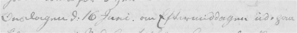Ondagen d. 16 Juni. om Eftermiddagen ude paa
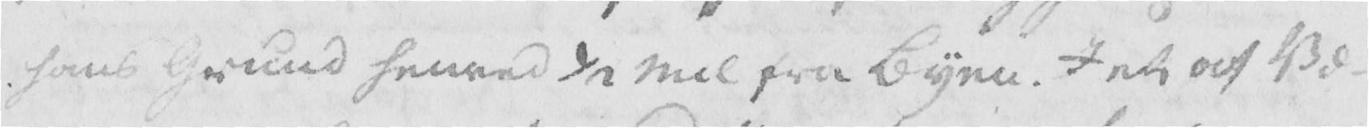hans Grund henved 1/2 Mil fra Byen. I et af Væ-
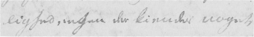lighed, ingen der kiender noget
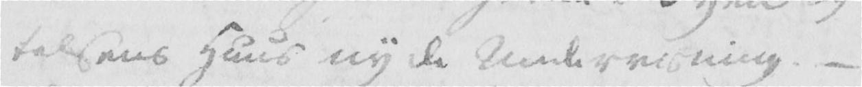telsens Huus nyde Undervisning. -
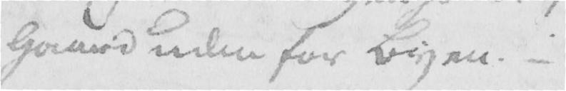Gaard uden for Byen. -
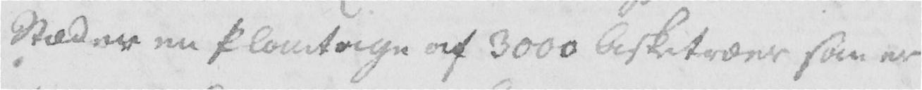Stæd er en Plantage af 3000 Asketræer som er
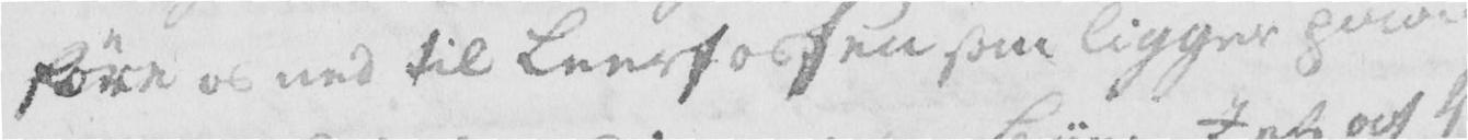føre os ned til Leerfossen som ligger paa
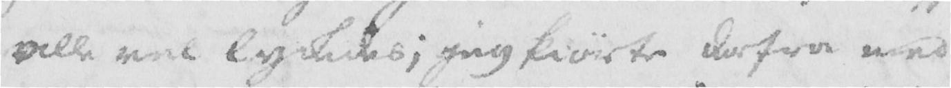alle vel lyckedes; jeg kiørte derfra med
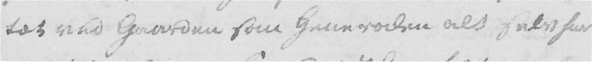tæt ved Gaarden som Generalen alt selv har
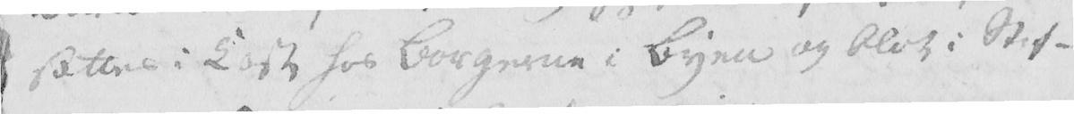sættes i Kost hos Borgerne i Byen og blot i Stif-
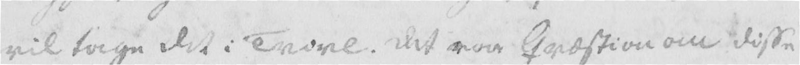vil tage det i Tvivl. Det var Qvæstion om disse
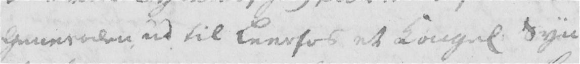Generalen ud til Leerfos et Kongel. Syn
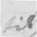til
